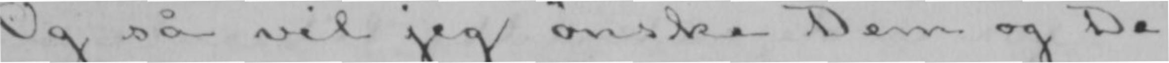Og så vil jeg ønske Dem og De-
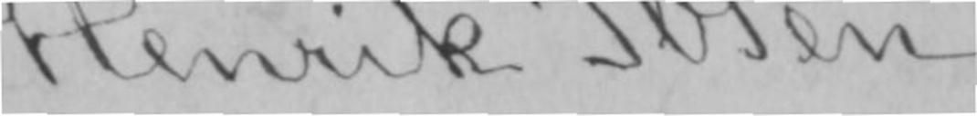Henrik Ibsen.
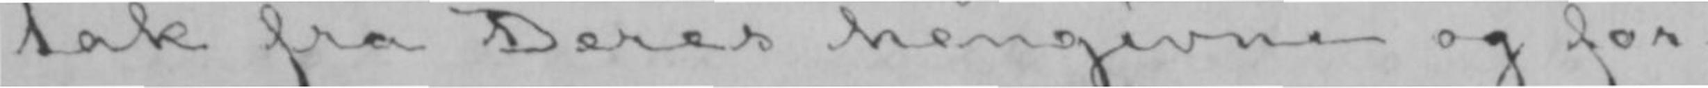tak fra Deres hengivne og for-
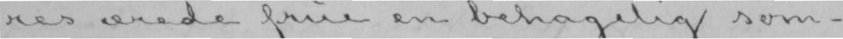res ærede frue en behagelig som-
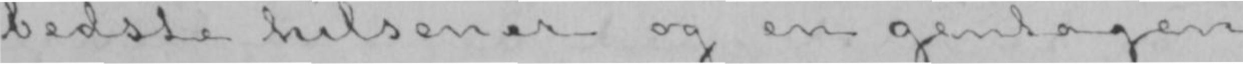bedste hilsener og en gentagen
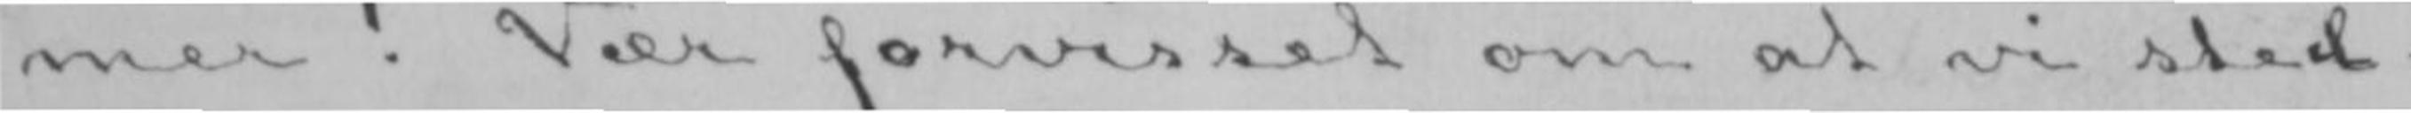mer! Vær forvisset om at vi sted-
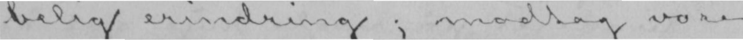belig erindring; modtag vore
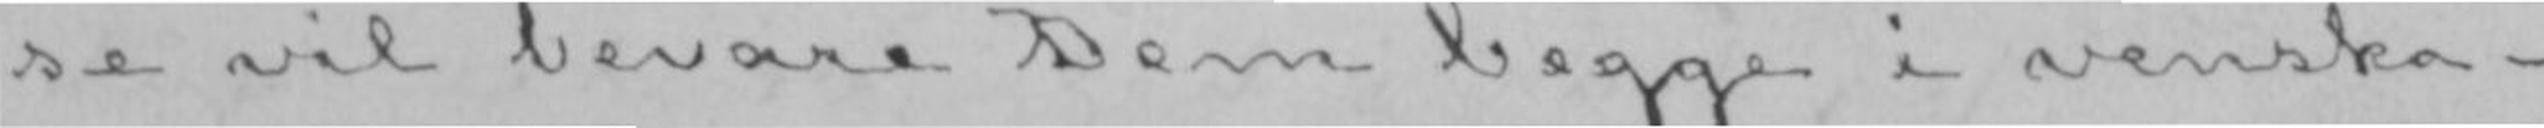se vil bevare Dem begge i venska-
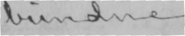bundne
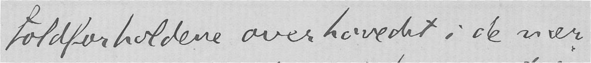toldforholdene overhovedet i de nær-
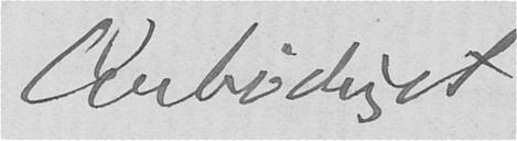Ærbødigst
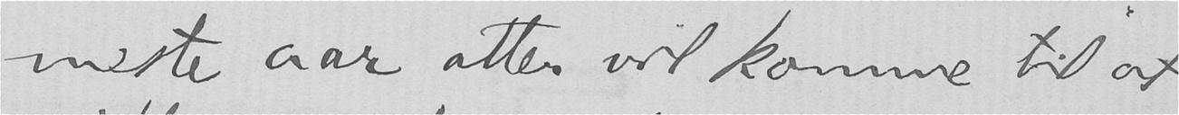meste aar atter vil komme til at
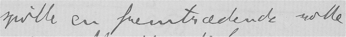spille en fremtrædende rolle
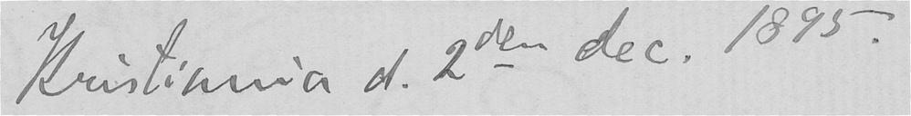Kristiania d. 2den dec. 1895.
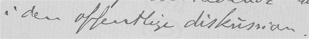i den offentlige diskussion.
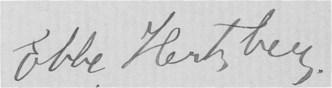Ebbe Hertzberg.
